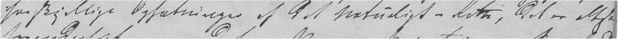forskjellige Opfatninger af det Naturligt Rette, det er altsaa
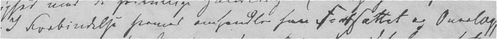I Forbindelse hermed omhandler han Forsættet og Overlægg,
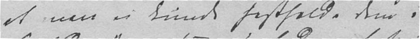at man ei kunde fastholde dem i
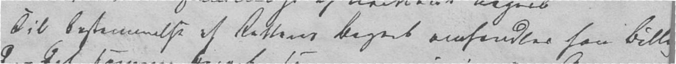Til Bestemmelse af Rettens Begreb omhandler han Billig-
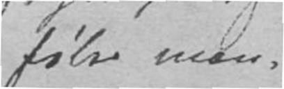føler man,
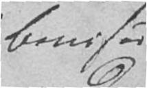Beviser
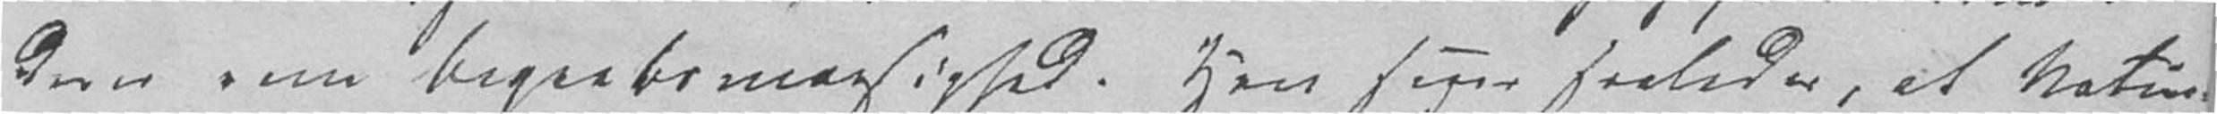deres rene Begrebsmæssighed. Han siger saaledes, at Natur-
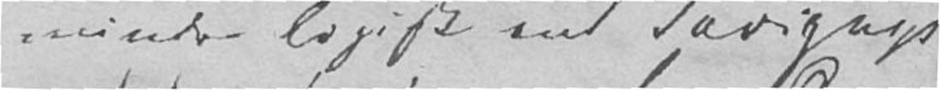mindre logisk end Savignys
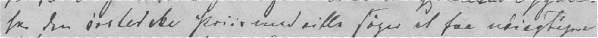for den ørstedske Priismedaille søger at faa nøiagtigere
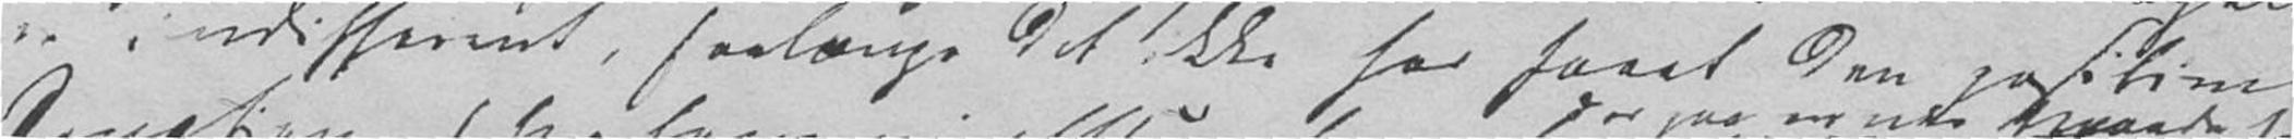er indifferent, saalænge det ikke har faaet den positive
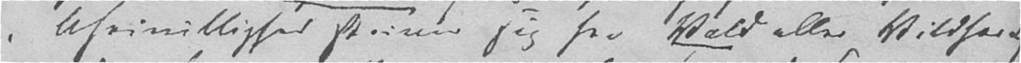. Ufrivillighed skriver sig fra Vold eller Vildfarelse
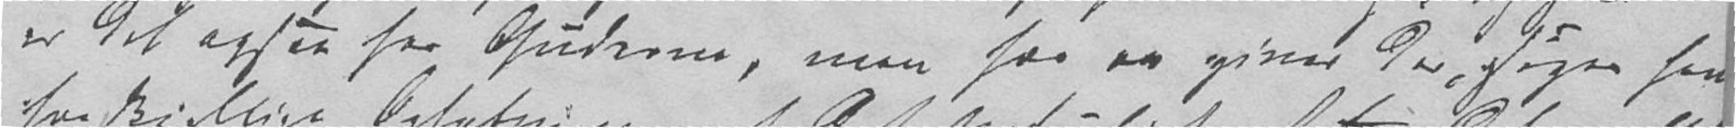er det ogsaa for Guderne, men hos os gives der, siger han,
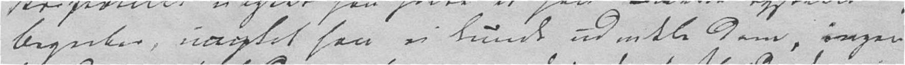Begreber, uagtet han ei kunde udvikle dem, ingen-
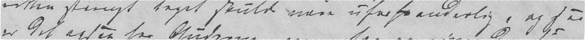retten strengt taget skulde være uforfoanderlig, og saa
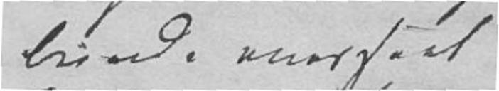lunde overseet
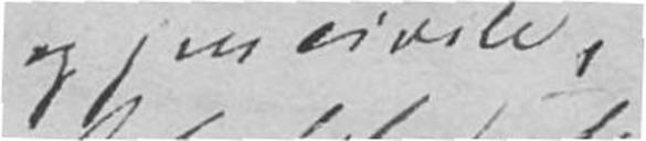og jus civile,
